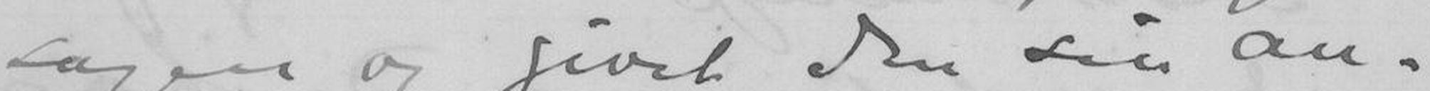sagen og givet den sin an-
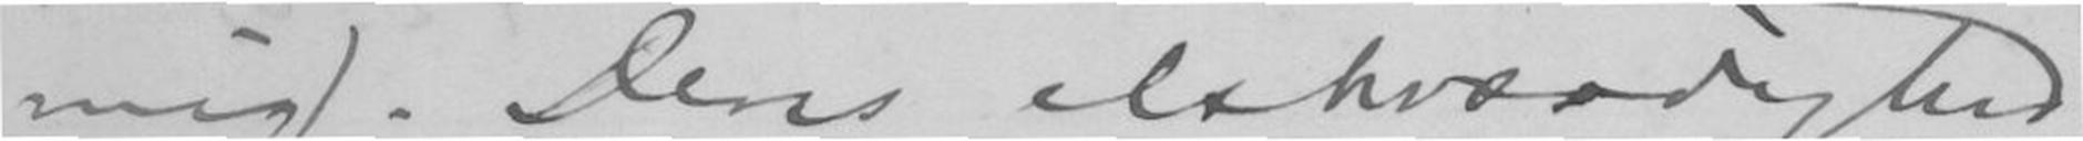mig. Deres elhværdighed
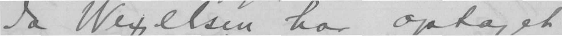da Wexelsen har optaget
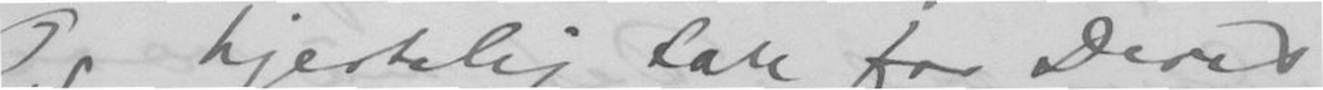Og hjertelig tak for Deres
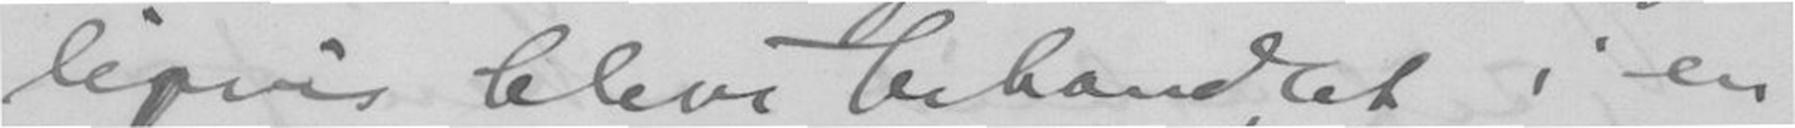ligvis blive behandlet i en
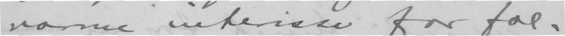varme interesse for fol-
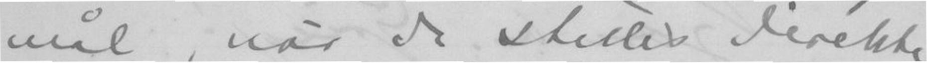smål, når de stilles direkte
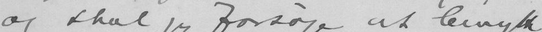og skal jeg forsøge at benytte
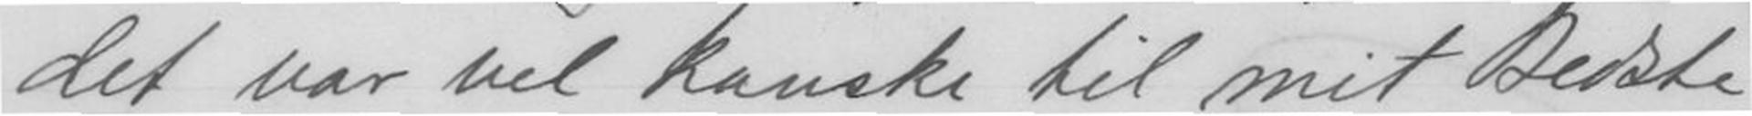det var vel kanske til mit Bedste
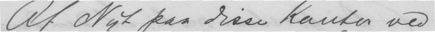Af Nyt paa disse kanter ved
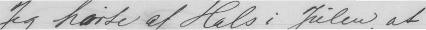Jeg hørte af Hals i Julen, at
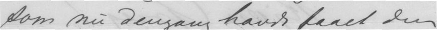som nu dengang havde faaet den
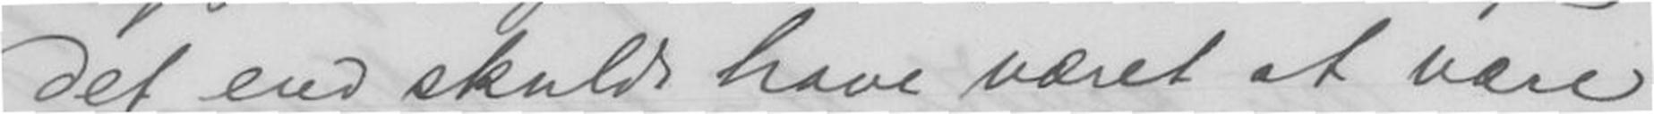det end skulde have været at være
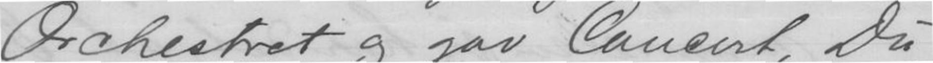Orchestret og gav Concert, Du
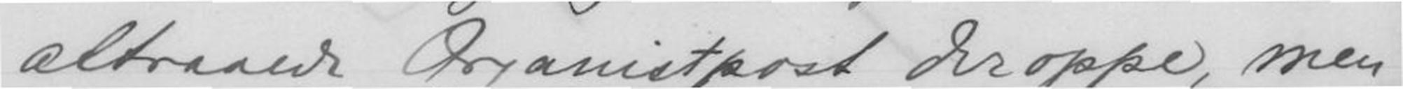altraaede Organistpost deroppe, men
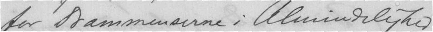for Drammenserne i Almindelighed
